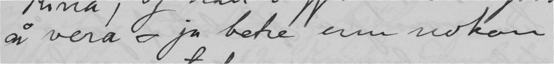å vera – ja betre enn nokon
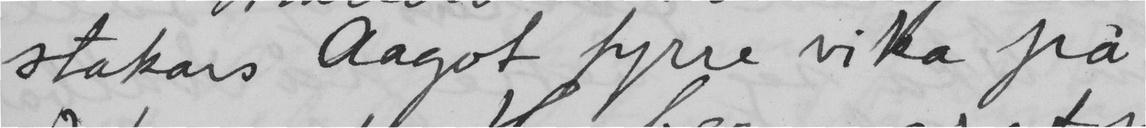stakars Aagot fyrre vika på
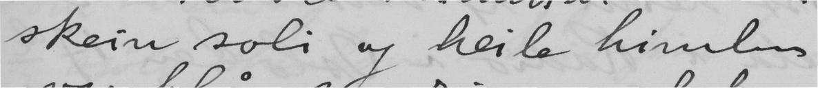skein soli og heile himlen
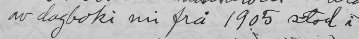av dagboki mi frå 1905 stod i
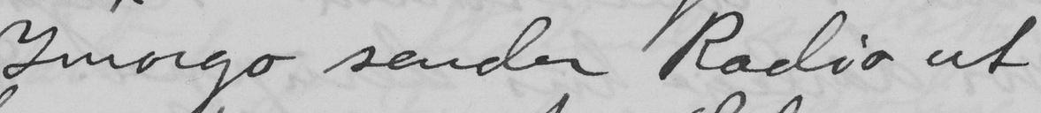Imorgo sender Radio eit
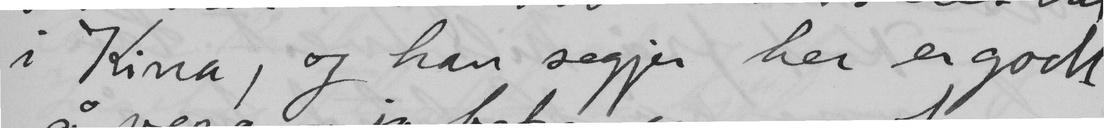i Kina, og han segjer her er godt
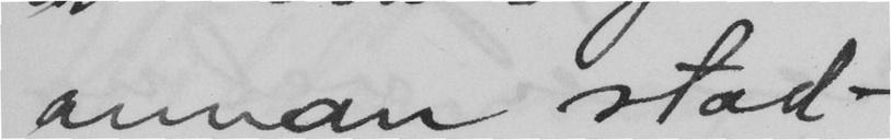annan stad –
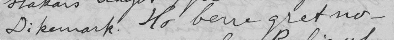Dikemark. Ho berre gret no –
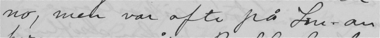no, men var ofte på Sin-an
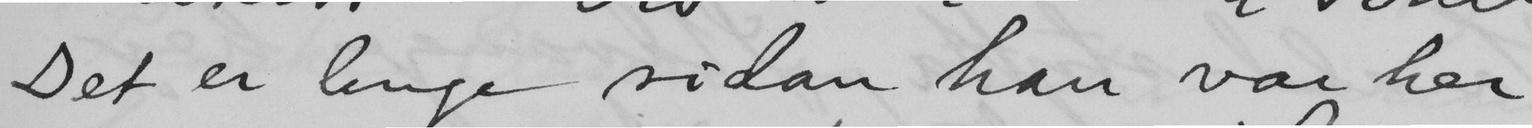Det er lenge sidan han var her
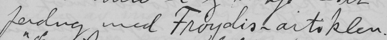ferdig med Fröydis-artiklen.
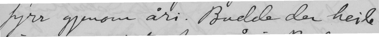fyrr gjenom åri. Budde der heile
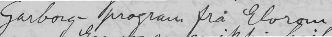Garborg-program frå Elvrum –
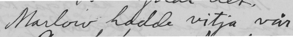Marlow hadde vitja vår
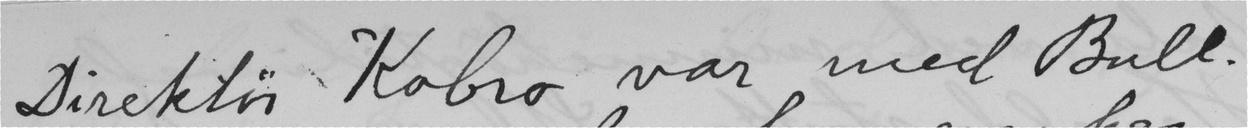Direktör Kobro var med Bull.
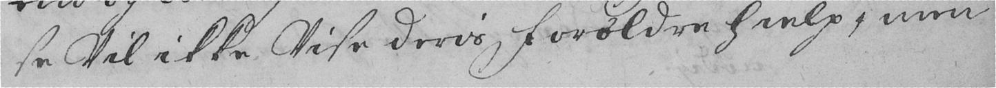se Vil ikke Vise deris Forældre hielp, men
